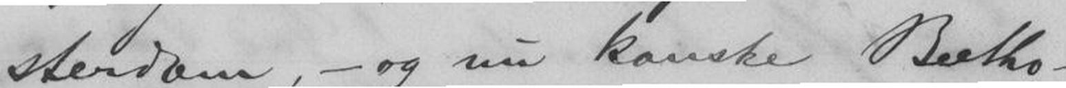sterdam, - og nu kanske Beetho-
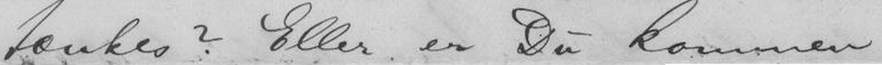tænkes? Eller er Du kommen
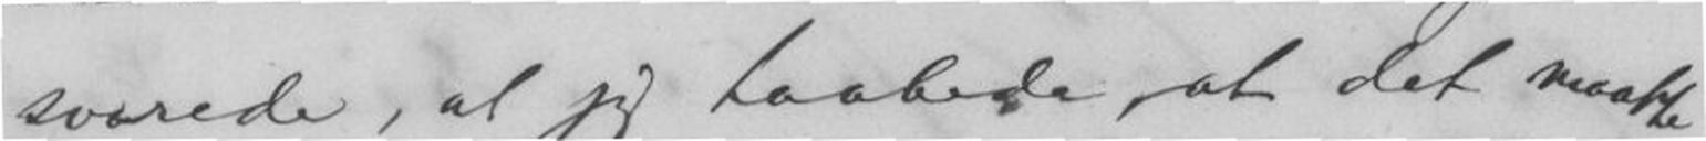svarede, at jeg haabede, at det maatte
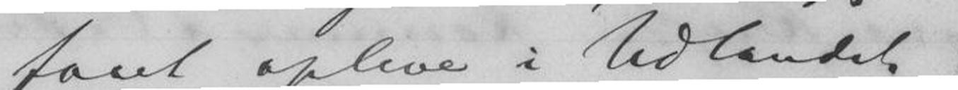faaet opleve i Udlandet:
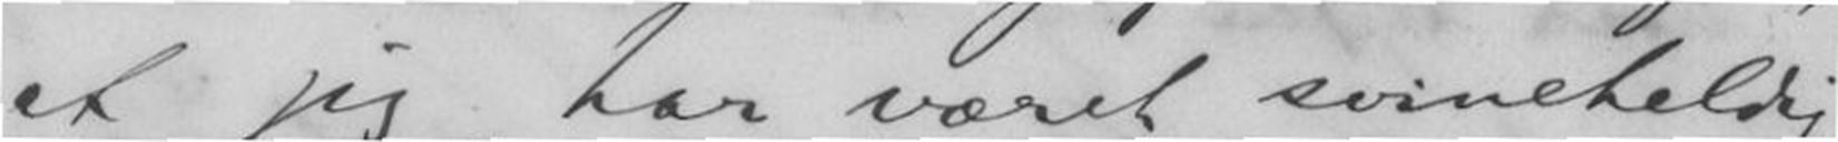at jeg har været svineheldig
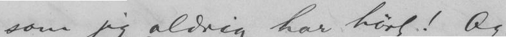som jeg aldrig har hørt! Og
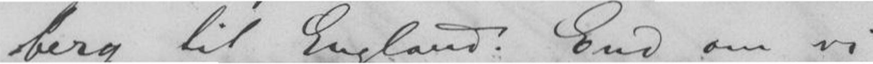berg til England. End om vi
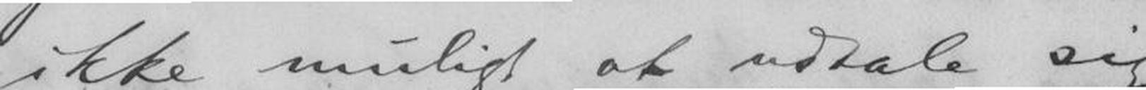ikke muligt at udtale sig
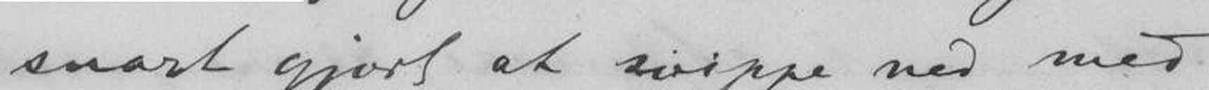snart gjort at svippe ned med
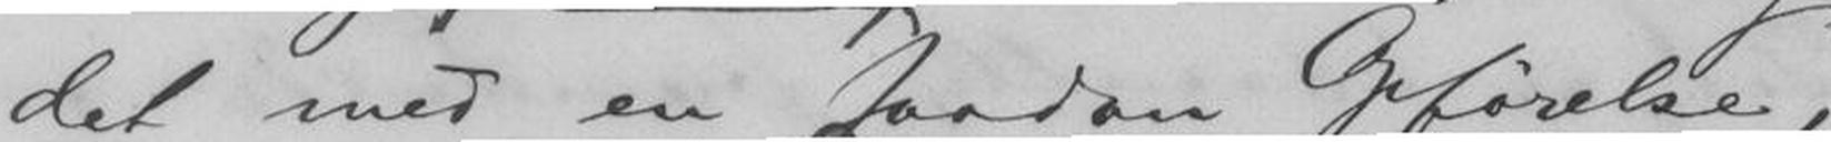det med en saadan Opførelse,
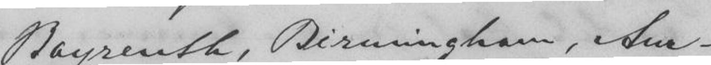Bayreuth, Birmingham, Am-
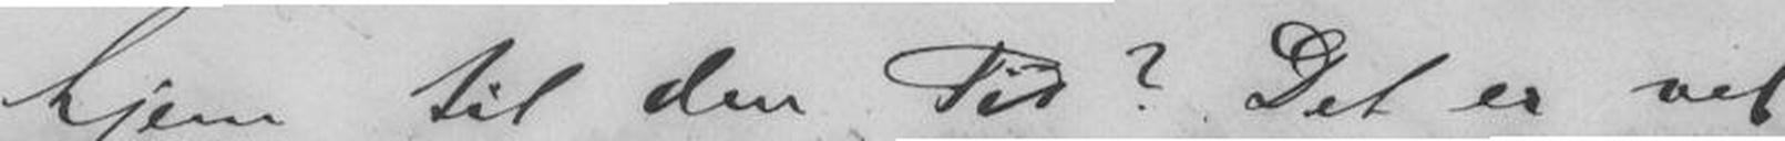hjem til den Tid? Det er vel
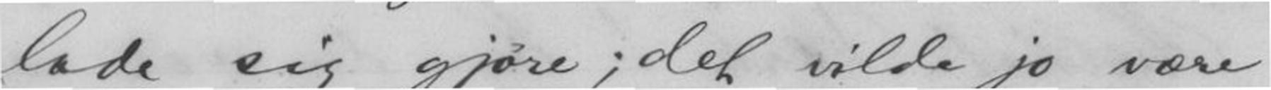lade sig gjøre; det vilde jo være
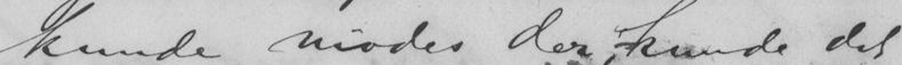kunde mødes der, kunde det
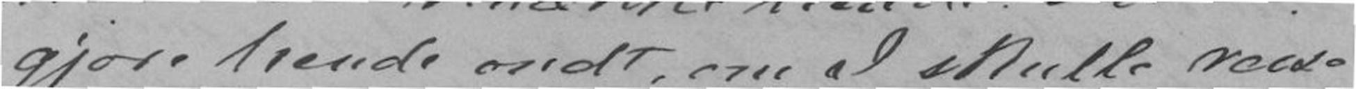gjøre hende ondt, om I skulde reise
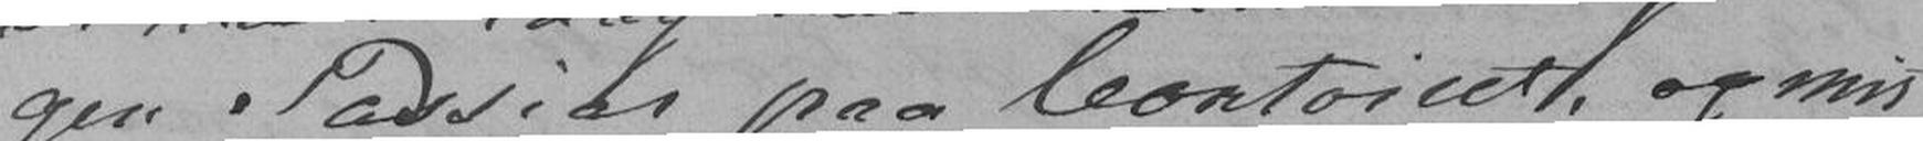gen Passiar paa Contoiret. og mit
